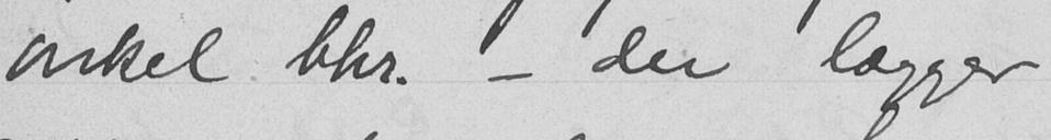onkel Chr. - der lægger
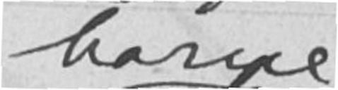barne
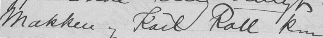Makken og Karl Roll kom
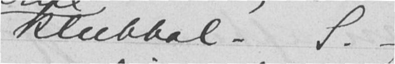klubbal. S. -
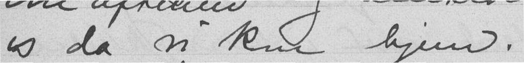os da vi kom hjem.
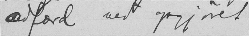adfærd ved opgjøret
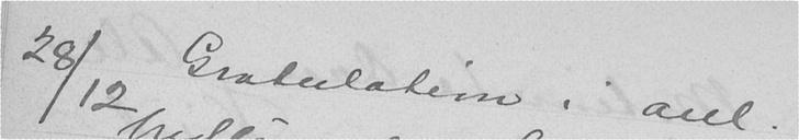28/12 Gratulation i anl.
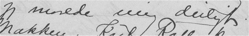jeg morede mig deiligt.
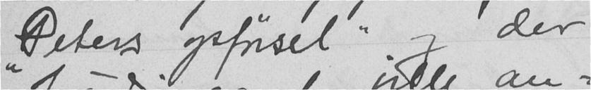"Peters opførsel" og der
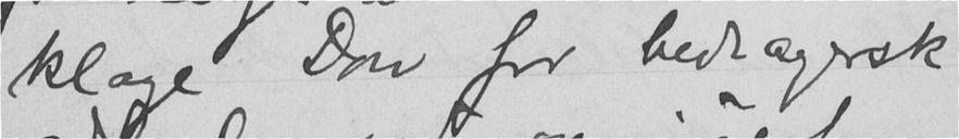klage Don for bedragersk
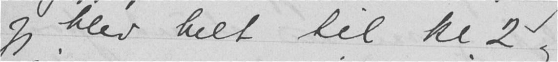jeg blev helt til kl 2,
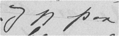og jeg paa
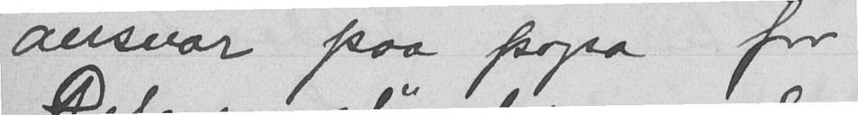ansvar paa papa for
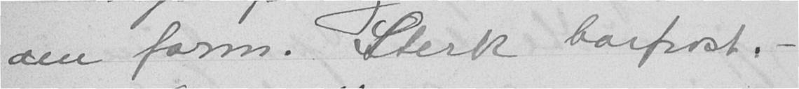om form. Sterk barfrost. -
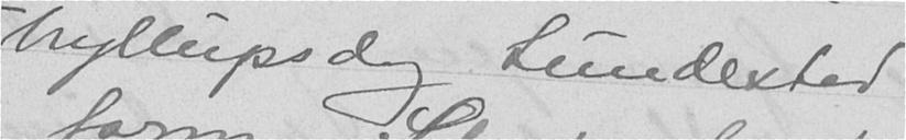bryllupsdag Lundestad
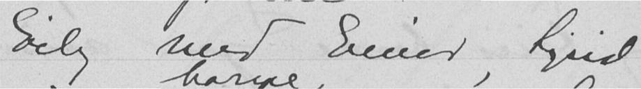Eilg med Einar, Sigrid
